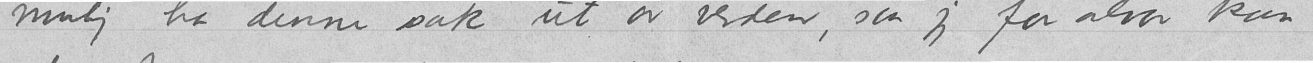mulig ha denne sak ut av verden, saa jeg for alvor kan
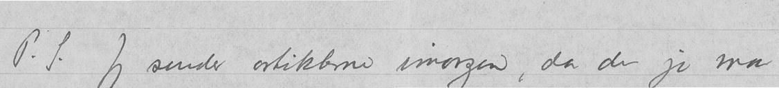P.S. Jeg sender artiklerne imorgen, da de jo maa
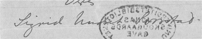Sigrid Undset Svarstad.
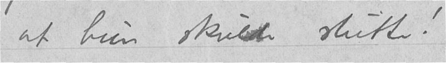at hun skulde slutte!
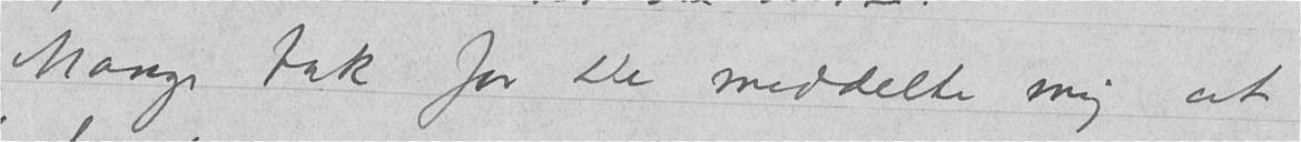Mange tak for De meddelte mig at
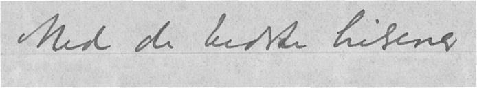Med de bedste hilsener
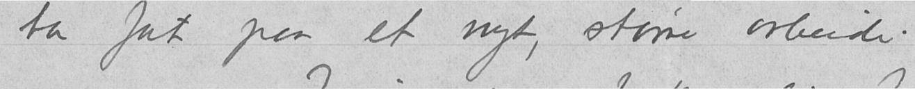ta fat paa et nyt, større arbeide.
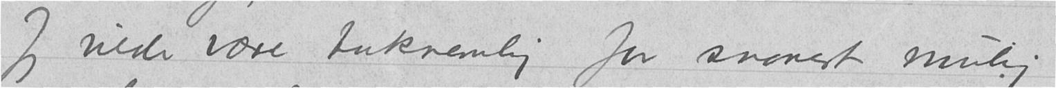Jeg vilde være taknemlig for snarest mulig
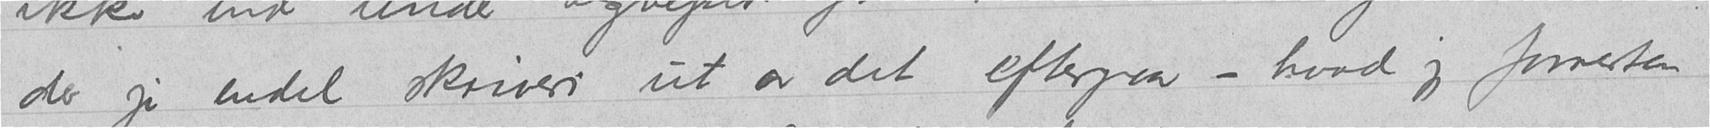der jo endel skriveri ut av det efterpaa – hvad jeg forresten
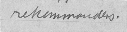rekommanderes.
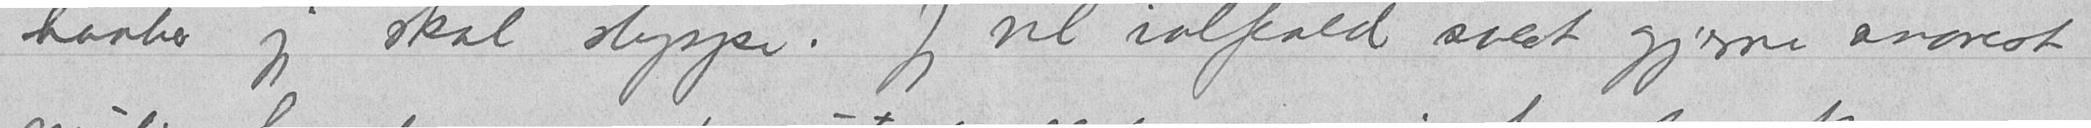haaber jeg skal slippe. Jeg vil ialfald svært gjerne snarest
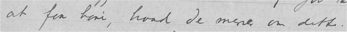at faa høre, hvad De mener om dette.
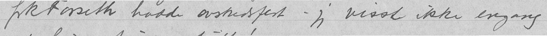frk Forseth hadde avskedsfest - jeg visste ikke engang
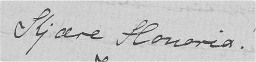Kjære Honoria!
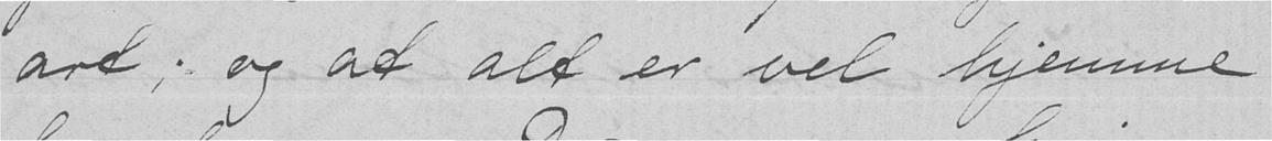art; og at alt er vel hjemme
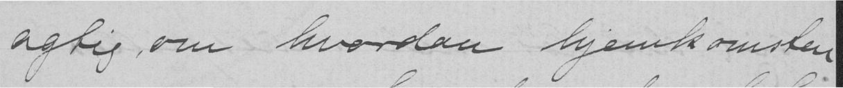agtig, om hvordan hjemkomsten
